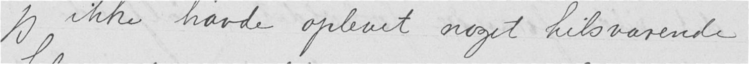jeg ikke havde oplevet noget tilsvarende
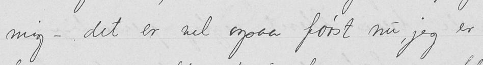mig - det er vel ogsaa først nu, jeg er
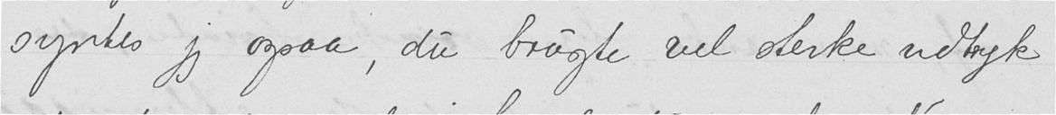syntes jeg ogsaa, du brugte vel sterke udtryk
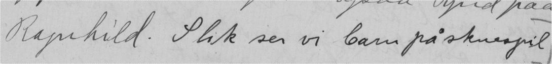Ragnhild. Slik ser vi barn på skuespil.
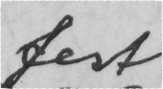fest
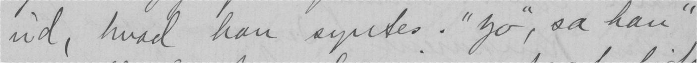ud, hvad han syntes. "Jo", sa han "
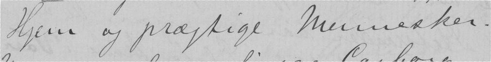Hjem og prægtige mennesker.
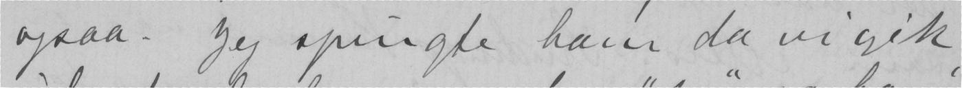ogsaa. Jeg spurgte ham da vi gik
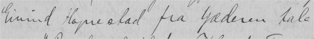Eivind Hognestad fra Jæderen tale
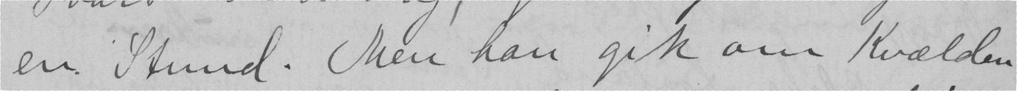en Stund. Men han gik om Kvælden
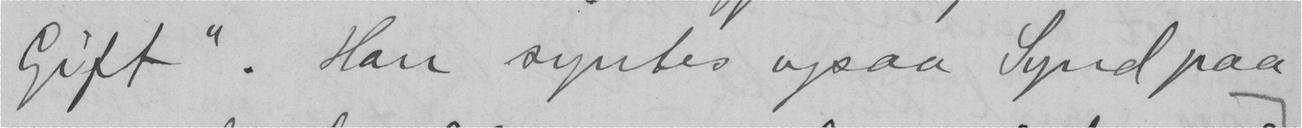Gift." Han syntes ogsaa Synd paa
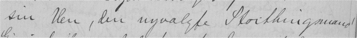sin ven, den nyvalgte Storthingsmand
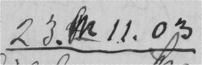23.  11. 03
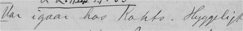Var igaar hos Kohts. Hyggeligt
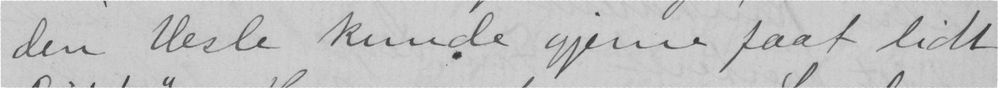den vesle kunde gjerne faat lidt
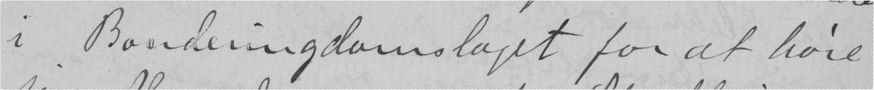i Bondeungdomslaget for at høre
